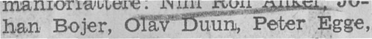han Bojer, Olav Duun, Peter Egge,
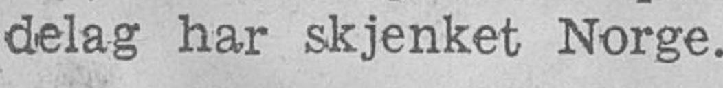delag har skjenket Norge.
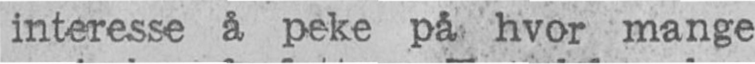interesse å peke på hvor mange
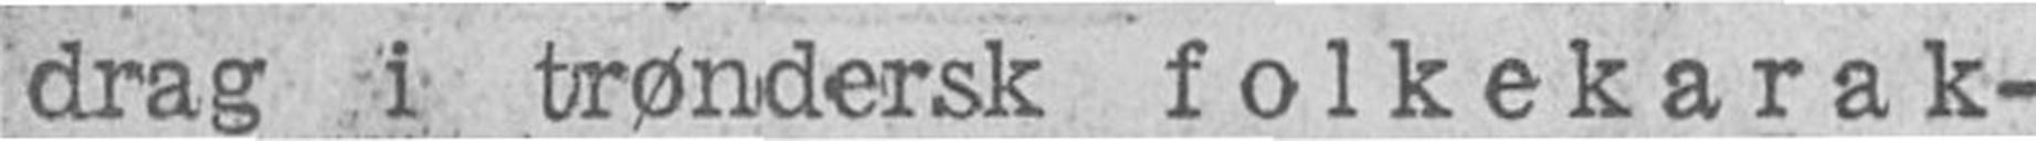drag i trøndersk folkekarak-
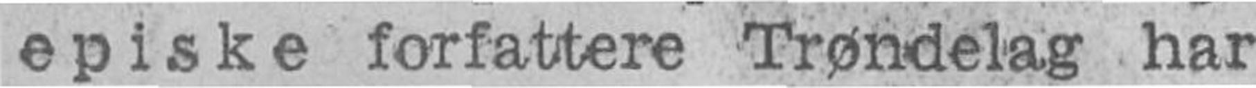episke forfattere Trøndelag har
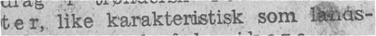ter, like karakteristisk som lands-
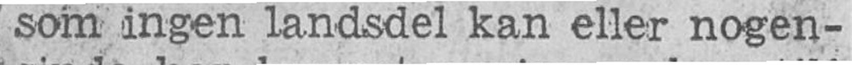som ingen landsdel kan eller nogen-
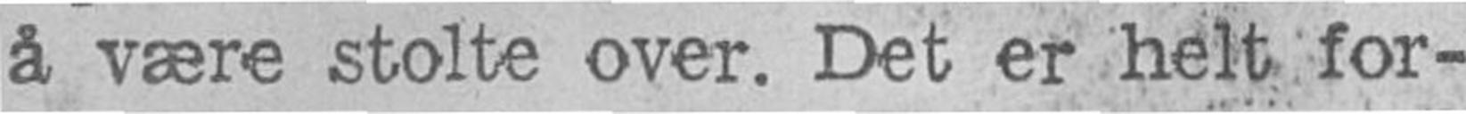å være stolte over. Det er helt for-
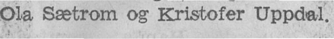Ola Sætrom og Kristofer Uppdal.
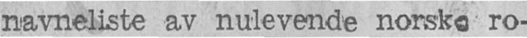navneliste av nulevende norske ro-
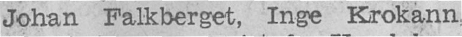Johan Falkberget, Inge Krokann,
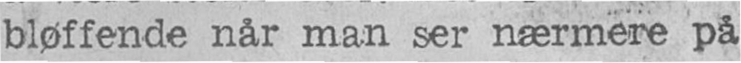bløffende når man ser nærmere på
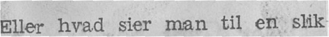Eller hvad sier man til en slik
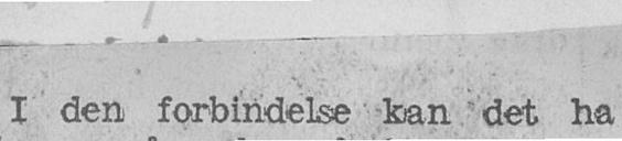I den forbindelse kan det ha
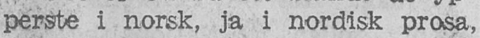perste i norsk, ja i nordisk prosa,
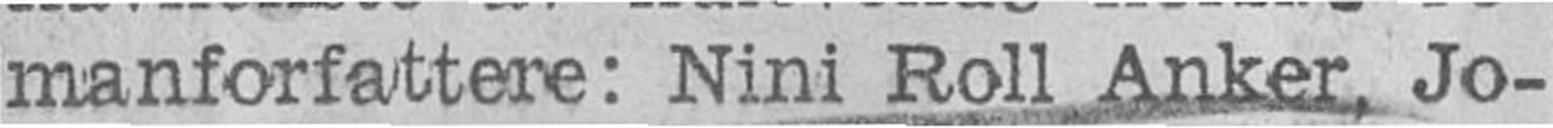manforfattere: Nini roll Anker, Jo-
